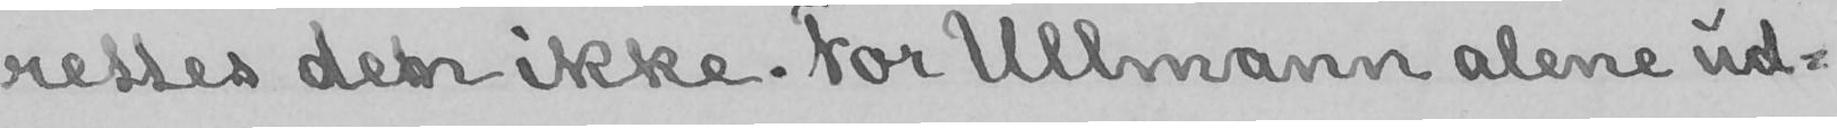rettes detn ikke. For Ullmann alene ud-
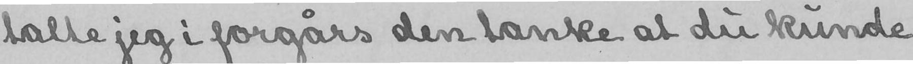talte jeg i forgårs den tanke at du kunde
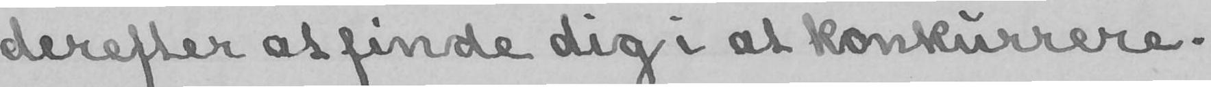derefter at finde dig i at konkurrere.
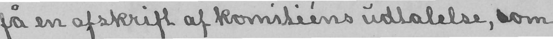få en afskrift af komiteéns udtalelse, som
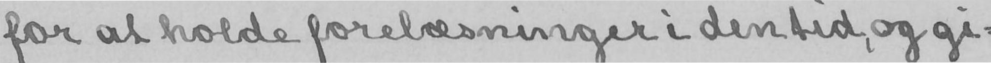for at holde forelæsninger i den tid, og gi-
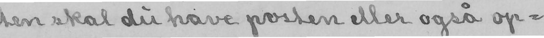ten skal du have posten eller også op-
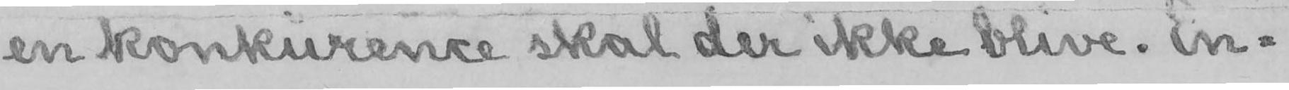en konkurence skal der ikke blive. En-
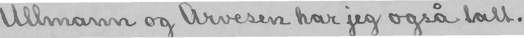Ullmann og Arvesen har jeg også talt.
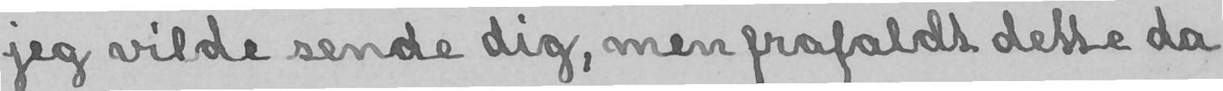jeg vilde sende dig, men frafaldt dette da
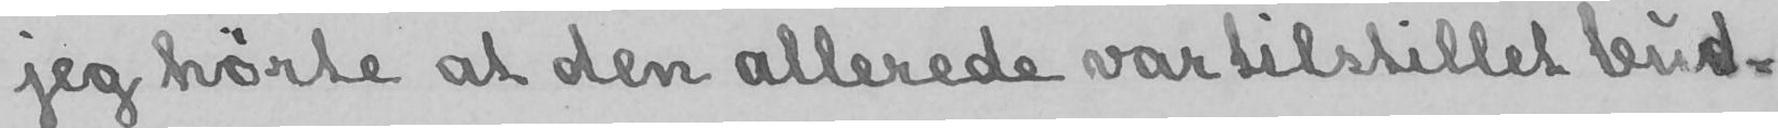jeg hørte at den allerede var tilstillet bud-
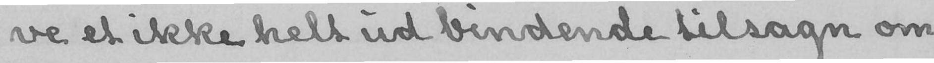ve et ikke helt ud bindende tilsagn om
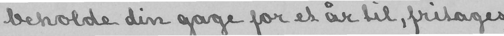beholde din gage for et år til, fritages
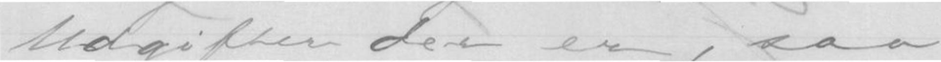Udgifter der er, saa
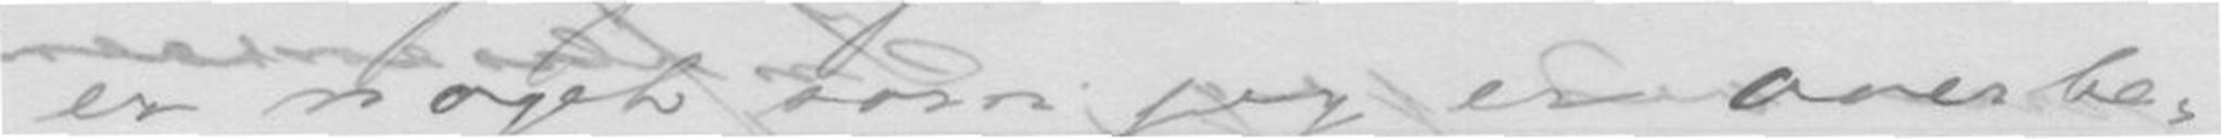er noget som jeg er overbe-
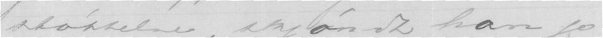støttelse, skjøndt han jo
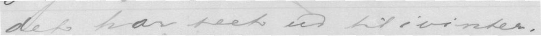det har seet ud til i vinter.
